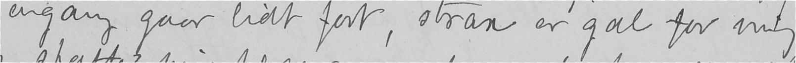engang gaar lidt fort, strax er gal for mig
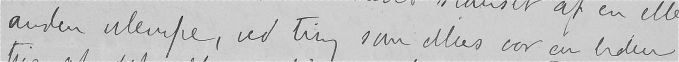anden ulempe, ved ting som ellers var en liden
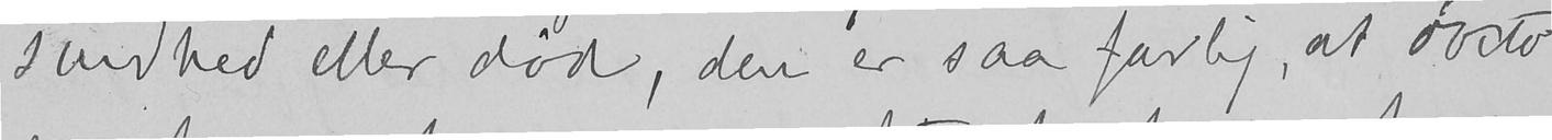sundhed eller død, den er saa farlig, at docto-
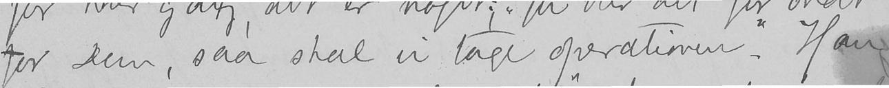for Dem, saa skal vi tage operationen." Han
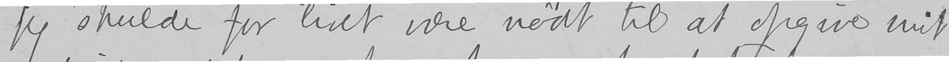jeg skulde for livet være nødt til at opgive mit
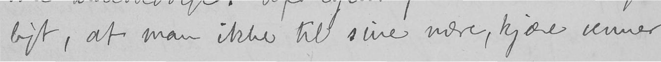ligt, at man ikke til sine nære, kjære venner
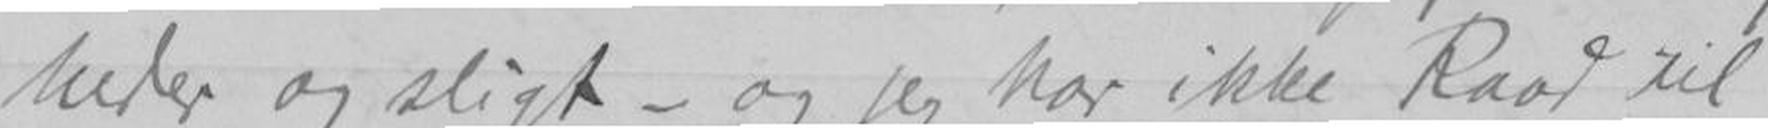heder og sligt - og jeg har ikke Raad til
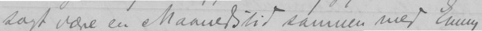sagt være en Maanedstid sammen med Emmy
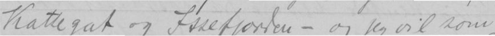Kattegat og Itsefjorden - og jeg vil som
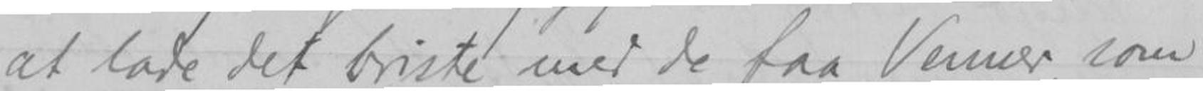at lade det briste med de faa Mennesker, som
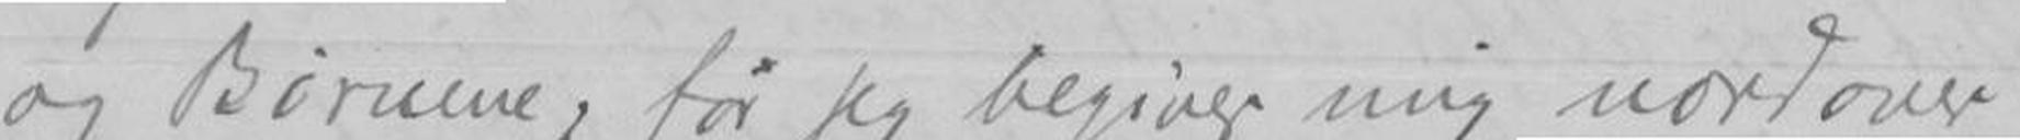og Børnene, før jeg begiver mig nordover
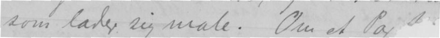som lader sig male. Om et Par
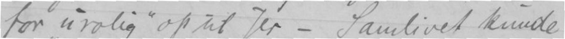for urolig op til Jer - Samlivet kunde
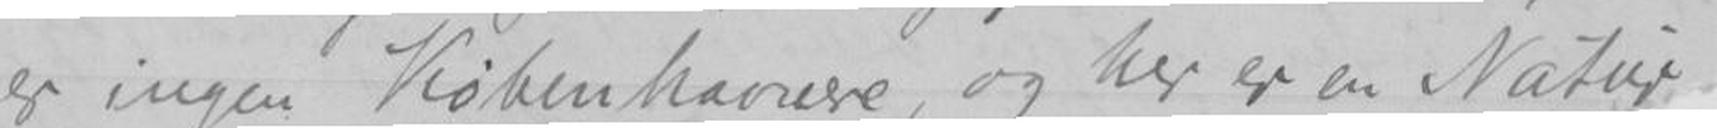er ingen Københavnere, og her er en Natur
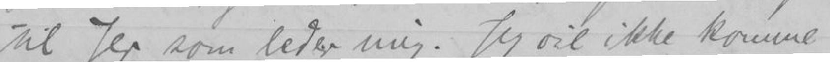til Jer som leder mig. Jeg vil ikke komme
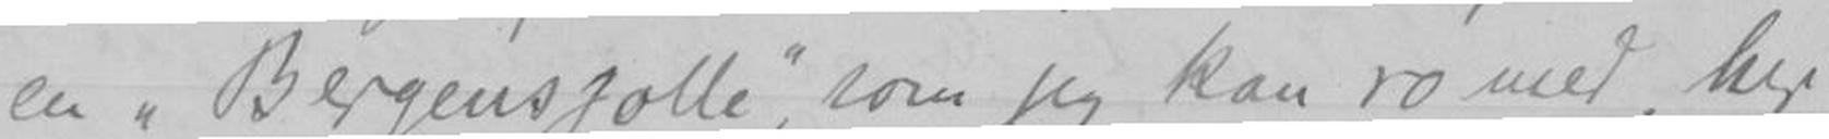en "Bergensjolle", som jeg kan ro med, her
